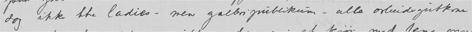dog ikke the ladies – men galleripublikum – alle arbeidergutterne
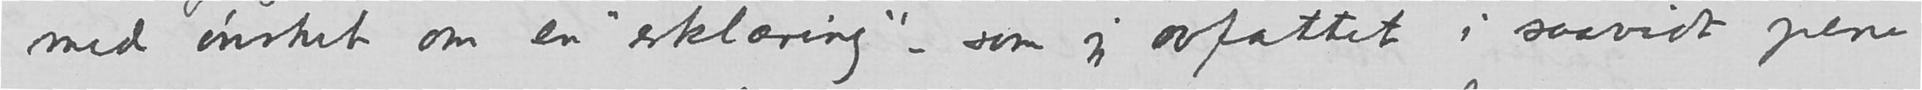med ønsket om en «erklæring» – som jeg avfattet i saavidt pene
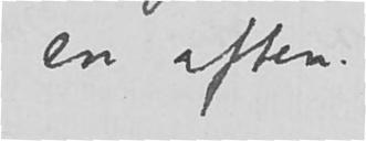en aften.
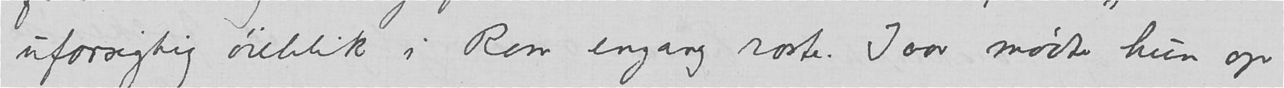uforsigtig øieblik i Rom engang roste. Iaar mødte hun op
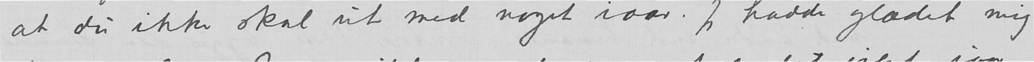at du ikke skal ut med noget iaar. Jeg hadde glædet mig
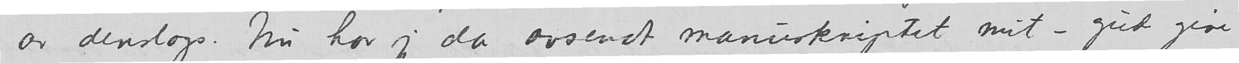av denslags. Nu har jeg da avsendt manuskriptet mit – gud give
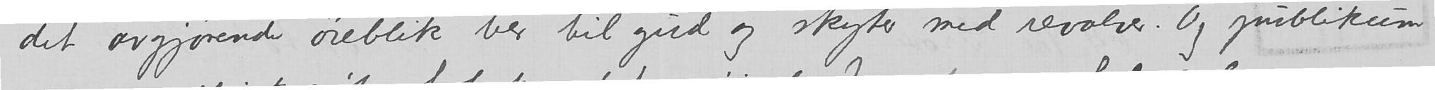det avgjørende øieblik ber til gud og skyter med revolver. Og publikum
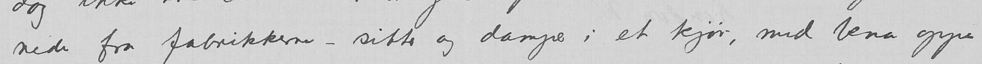nede fra fabrikkerne – sitter og damper i et kjør, med bena oppe
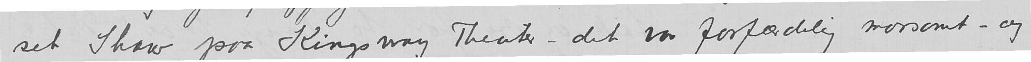set Shaw paa Kingsway Theater – det var forfærdelig morsomt – og
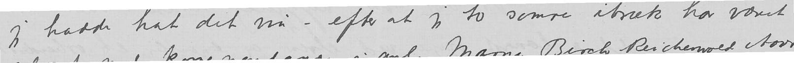jeg hadde hat det nu – efter at jeg to somre itræk har været
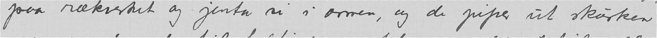paa rækverket og jenta si i armen, og de piper ut skurken
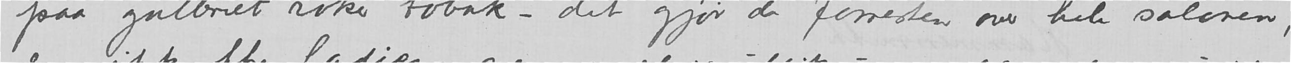paa galleriet røker tobak – det gjør de forresten over hele salonen
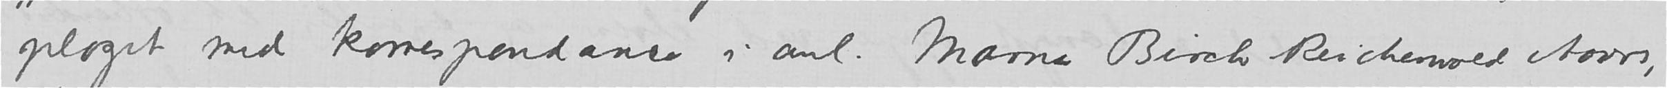ploget med korrespondance i anl. Marna Birch-Reichewald Aars,
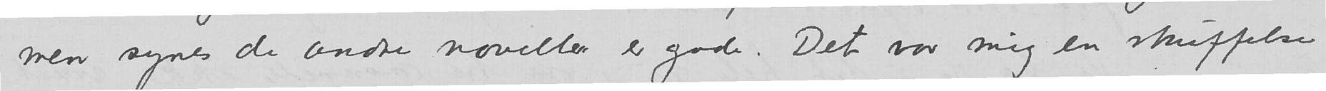men synes de andre noveller er gode. Det var mig en skuiffelse
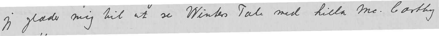jeg glæder mig til at se Winters Tale med Lilla Mc. Carthy
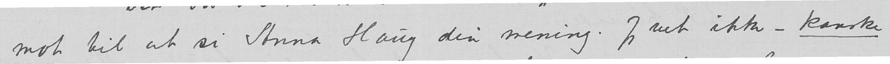mot til at si Anna Hang din mening. Jeg vet ikke – kanske
